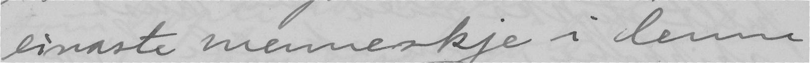einaste menneskje i denne
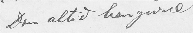Din altid hengivne
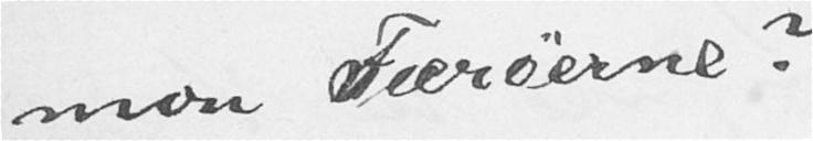mon Færøerne?
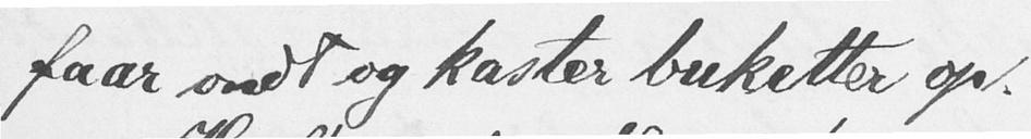faar ondt og kaster buketter op.
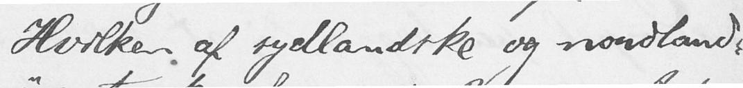Hvilken af sydlandske og nordland-
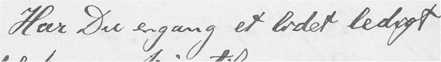Har Du engang et lidet ledigt
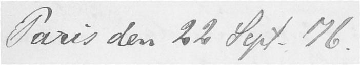Paris den 22 Sept. 76.
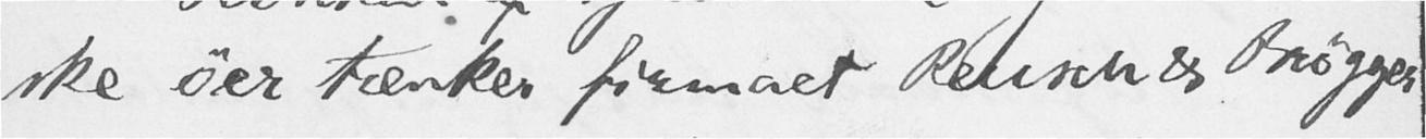ske øer tænker firmaet Reusch & Brøgger
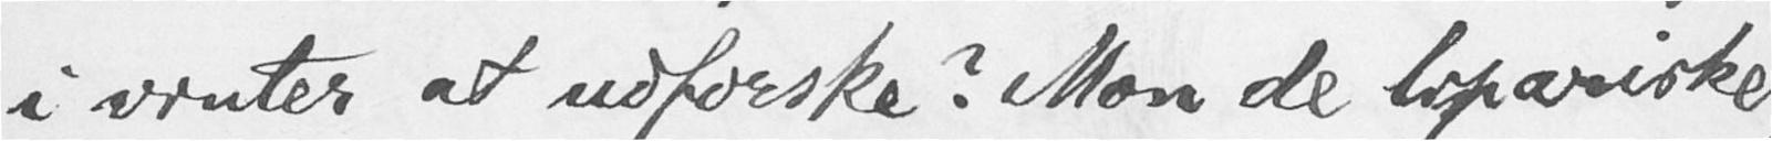i vinter at udforske? Mon de lipariske,
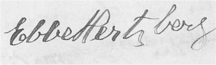Ebbe Hertzberg.
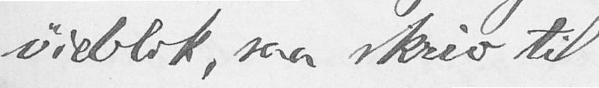øieblik, saa skrev til
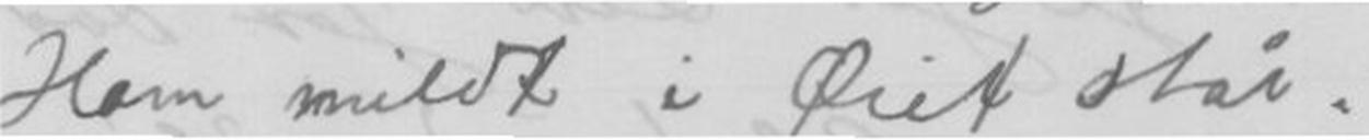Ham mildt i Øiet står.
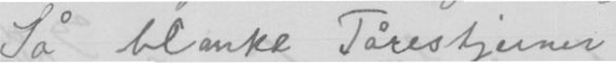Så blanke Tårestjerner
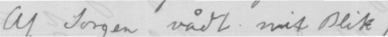Af Sorgen vådt mit Blik,
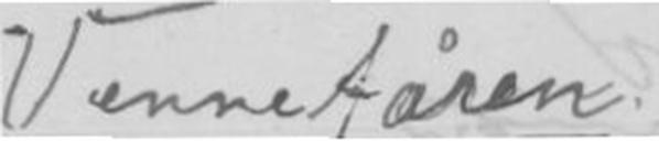* Vennetåren.
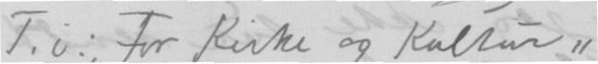Til: "For Kirke og Kultur"
